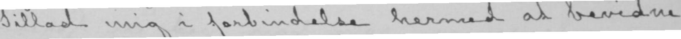Tillad mig i forbindelse hermed at bevidne
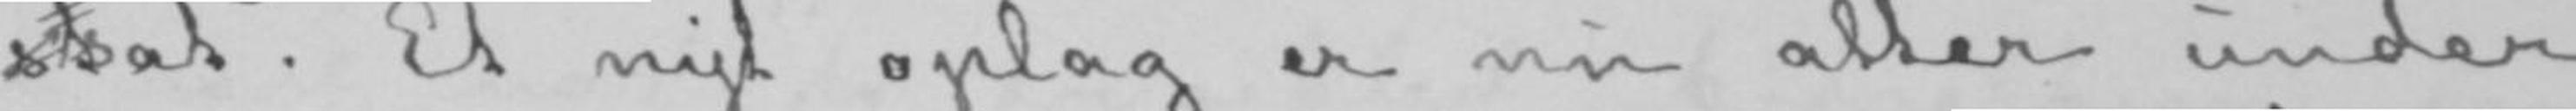stsat. Et nyt oplag er nu atter under
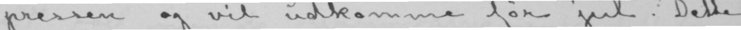pressen og vil udkomme før jul. Dette
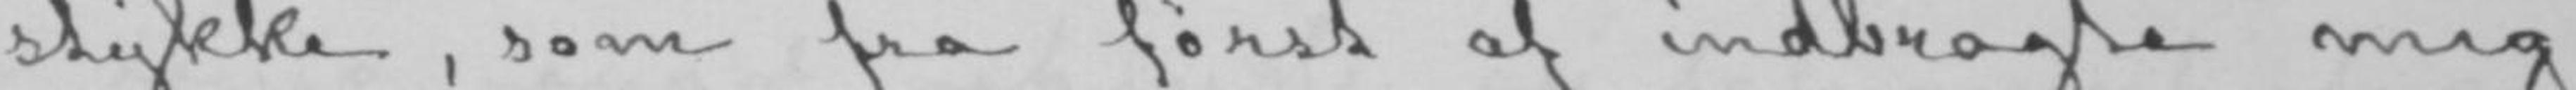stykke, som fra først af indbragte mig
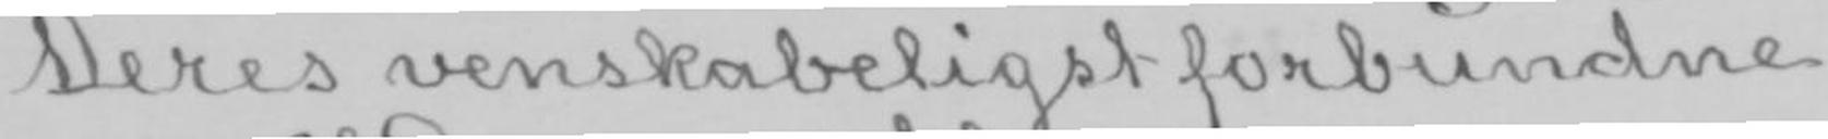Deres venskabeligst forbundne
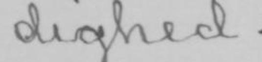dighed.
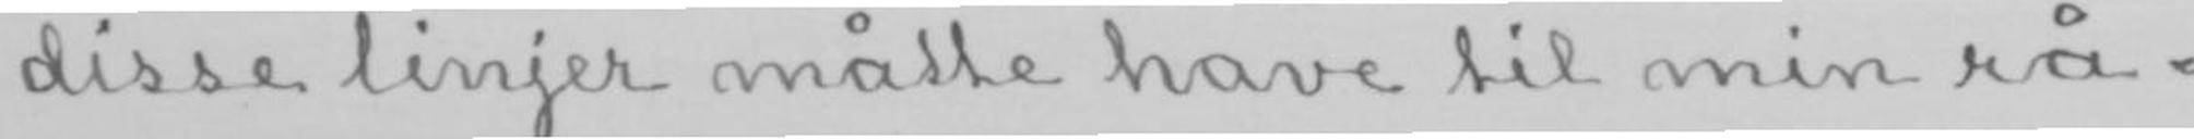disse linjer måtte have til min rå-
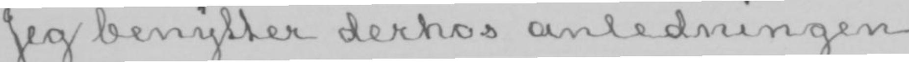Jeg benytter derhos anledningen
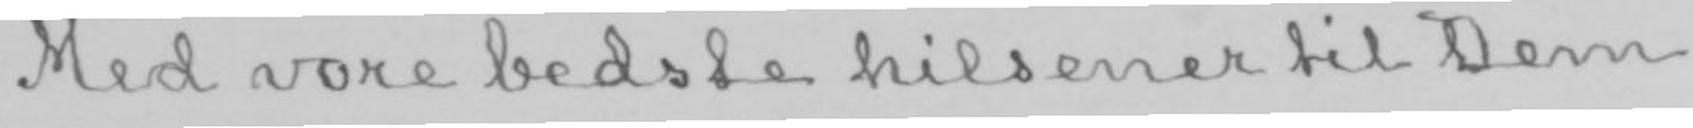Med vore bedste hilsener til Dem
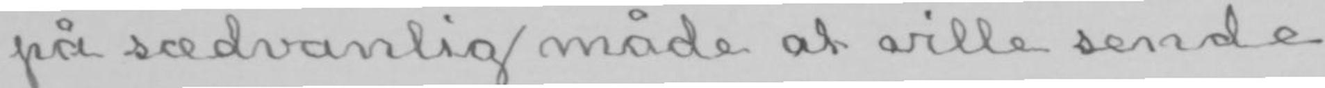på sædvanlig måde at ville sende
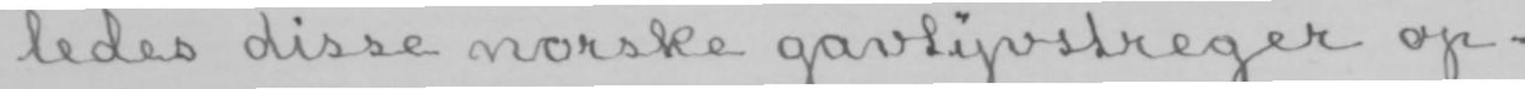ledes disse norske gavtyvstreger op-
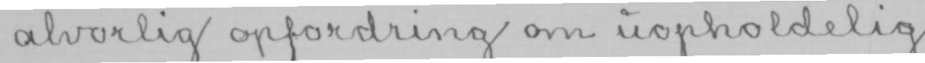alvorlig opfordring om uopholdelig
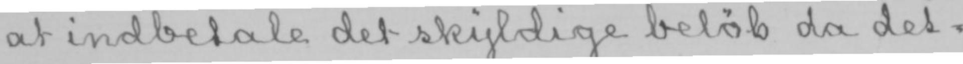at indbetale det skyldige beløb da det-
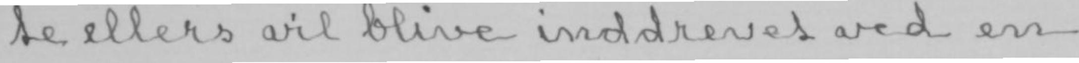te ellers vil blive inddrevet ved en
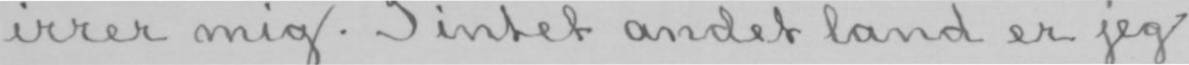irrer mig. I intet andet land er jeg
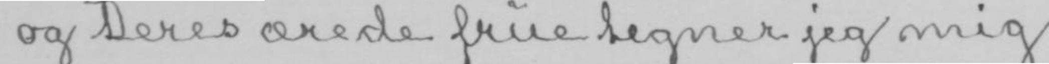og Deres ærede frue tegner jeg mig
